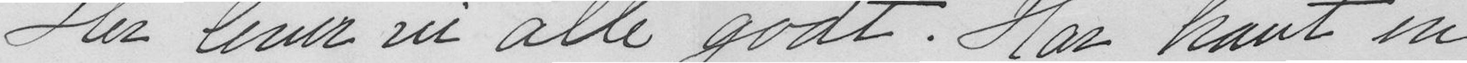Her lever vi alle godt. Har havt en
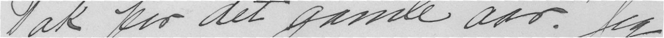Tak for det gamle aar. Jeg
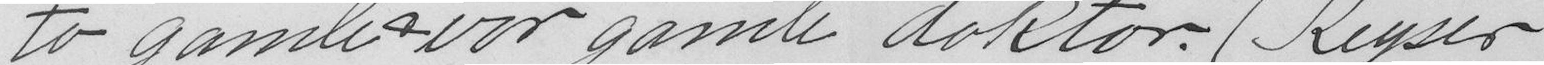to gamle & vor gamle doktor. (Keyser
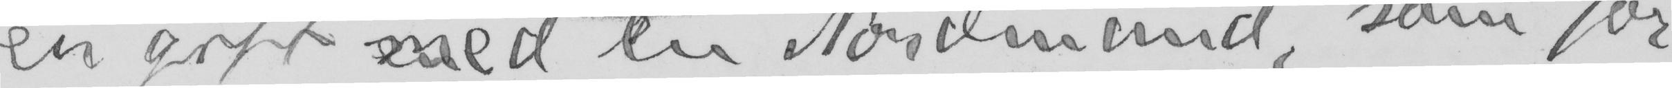er gift med en Nordmand, som for
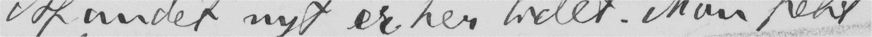Af andet nyt er her lidet. Mon petit
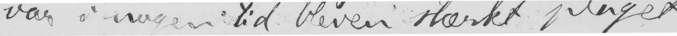var i nogen tid bleven stærkt plaget
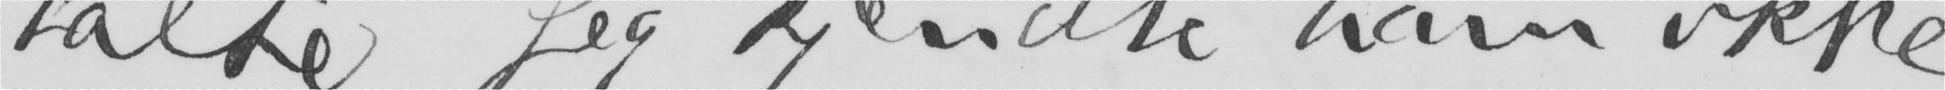talte. Jeg kjendte ham ikke.
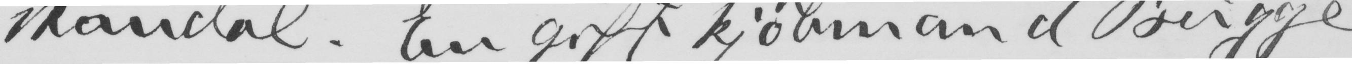skandal. En gift kjøbmand Bugge
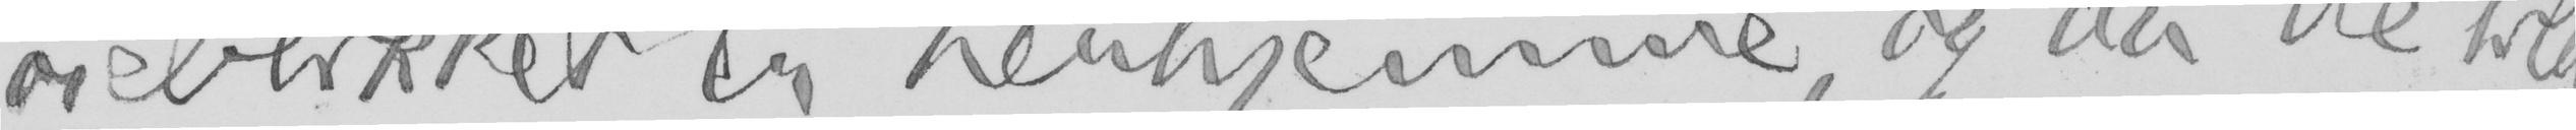øieblikket er herhjemme, og da de tillige
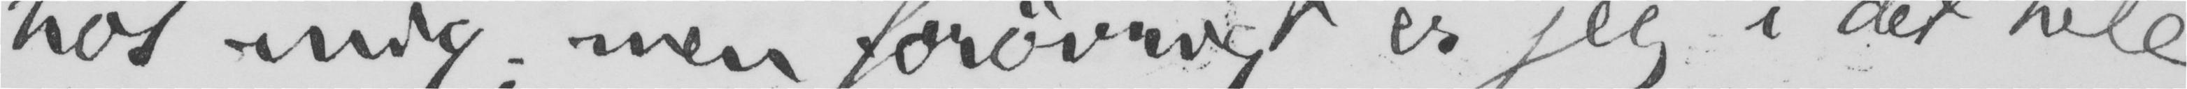hos mig; men forøvrigt er jeg i det hele
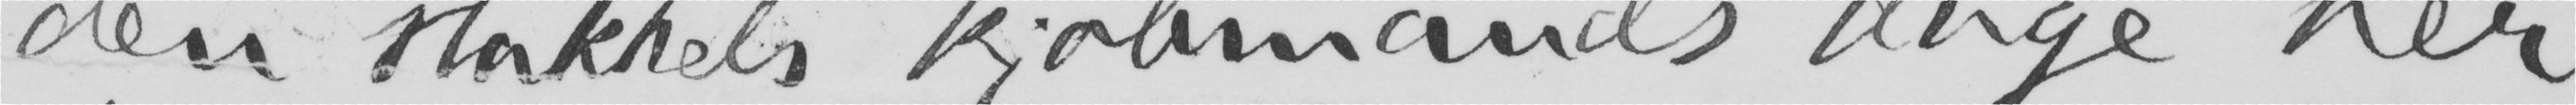den stakkels kjøbmands dage her-
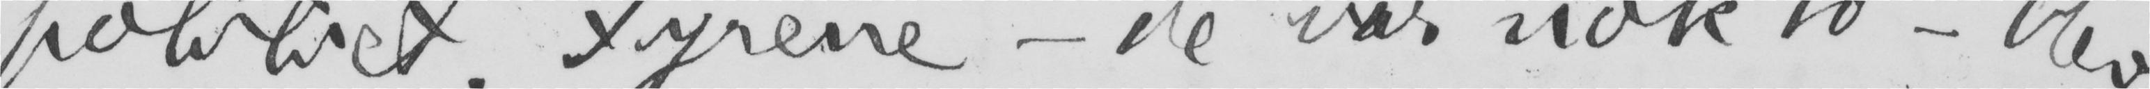politiet. Fyrene – de var nok to – blev
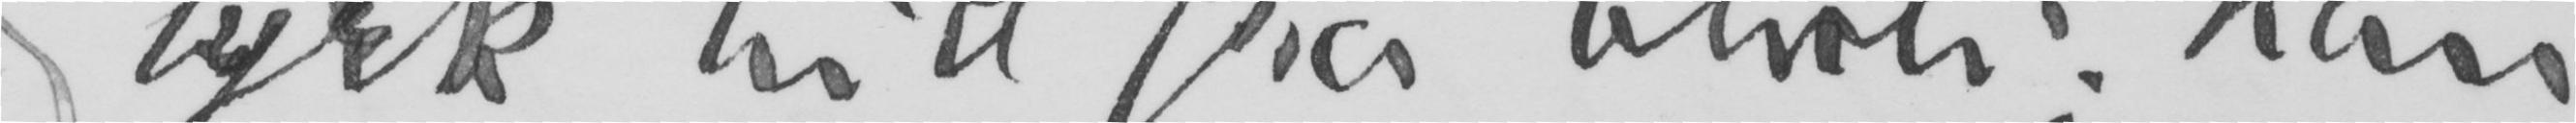Tyrk hid fra <…?>; han
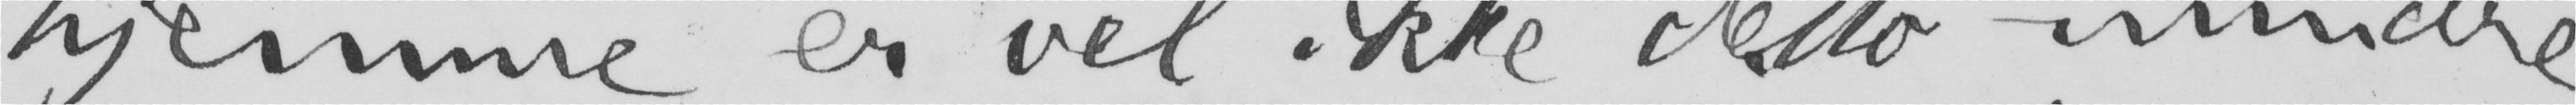hjemme er vel ikke desto mindre
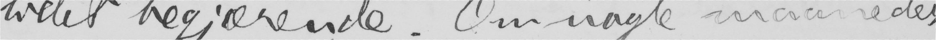lidet begjærende. Om nogle maaneder
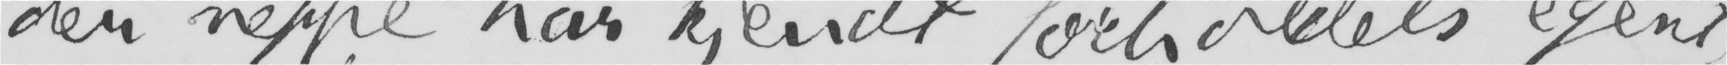der neppe har kjendt forholdets egent-
